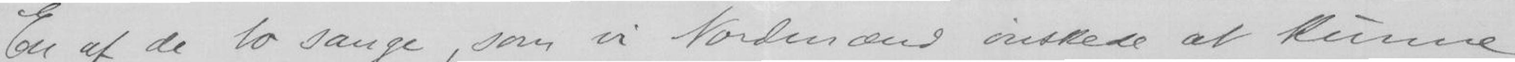En af de to sange, som vi Nordmænd ønskede at kunne
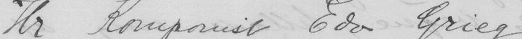Hr Komponist Edv Grieg
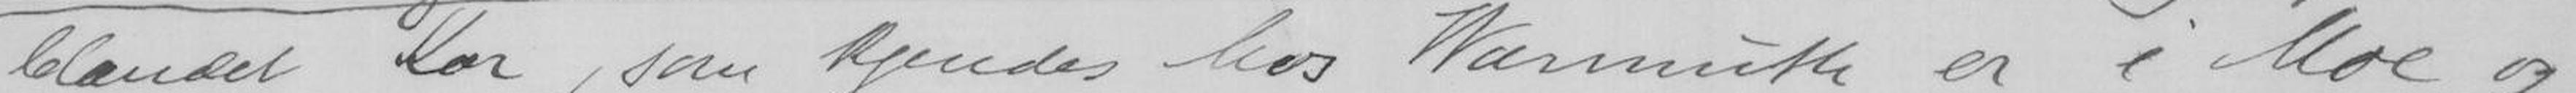blandet Kor, som kjendes hos Warnuth er i Moe og
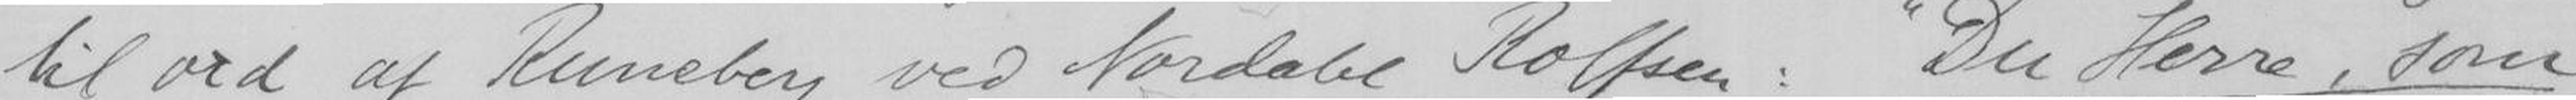til ord af Runeberg ved Nordahl Rolfsen. "Du herre, som
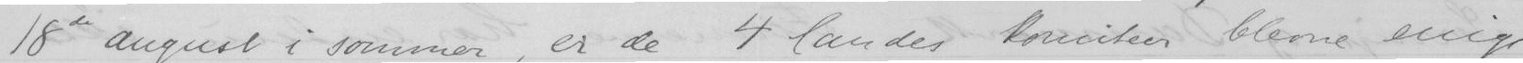18de august i sommer, er de 4 landes komiteen blevne enige
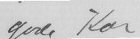gode Kor.
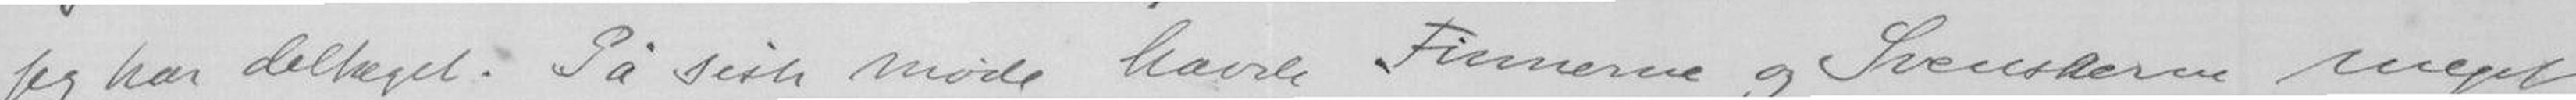jeg har deltaget. På siste møde havde Finnerne og Svenskerne meget
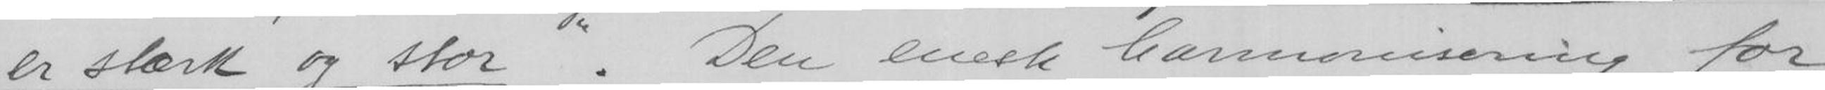er stærk og stor". Den eneste harmonisering for
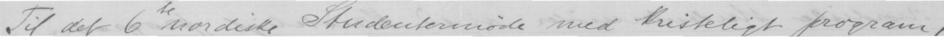Til det 6te nordiske Studentermøde med Kristeligt program,
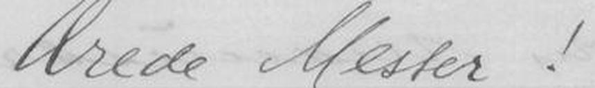Ærede Mester!
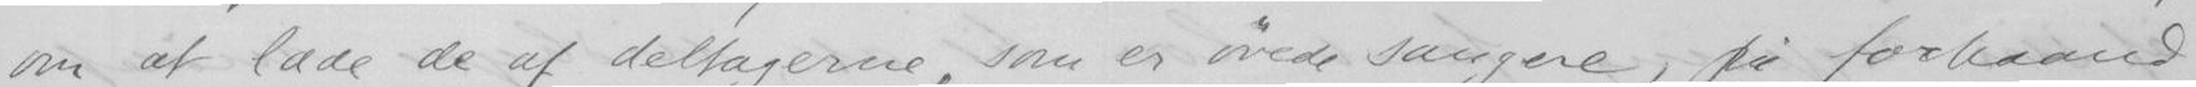om at lade de af deltagerne, som er øvede sangere, på forhaand
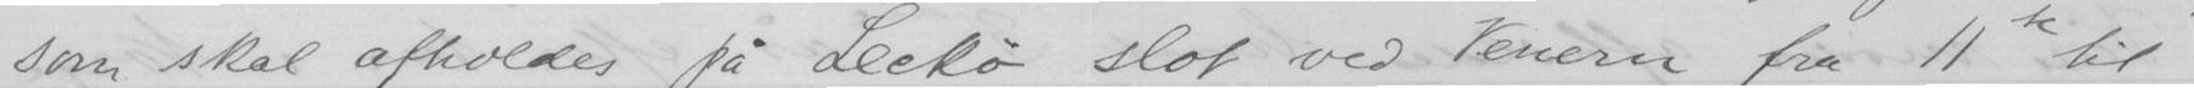som skal afholdes på Leckø slot ved Venern fra 11te til
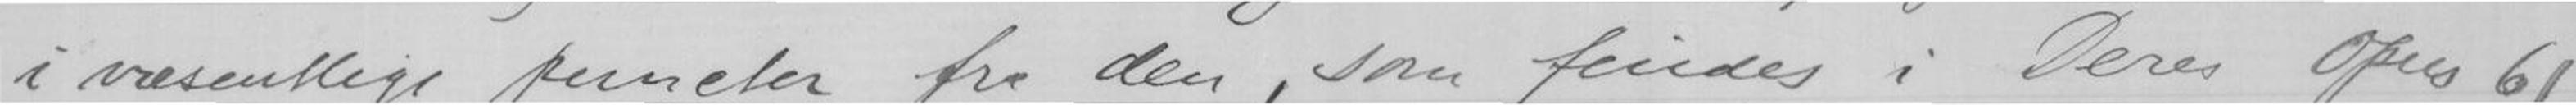i væsentlige puncter fra den, som findes i Deres Opus 61
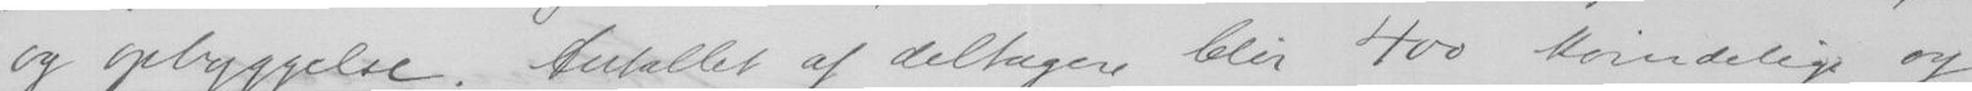og opbyggelse. Antallet af deltagere blir 400 kvindelige og
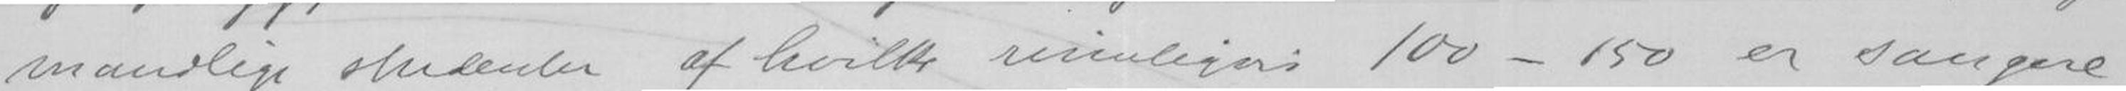mandlige studenter af hvilke rimeligvis 100 - 150 er sangere.
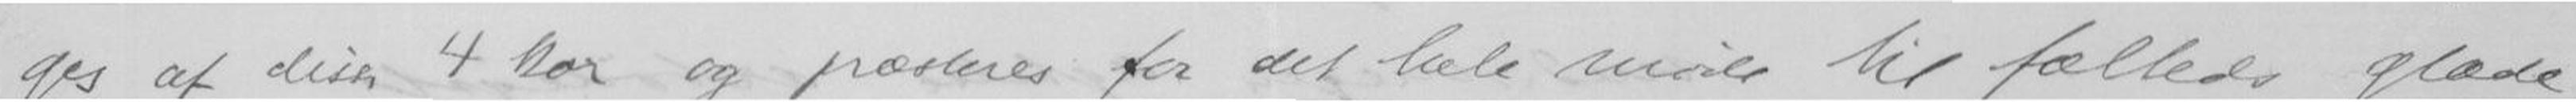ges af disse 4 kor og præsteres for det hele møde til fældes glæde
